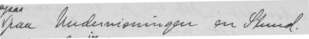paa Undervisningen en Stund.
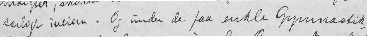særligt iveien. Og under de faa enkle Gymnastik-
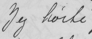Jeg hørte
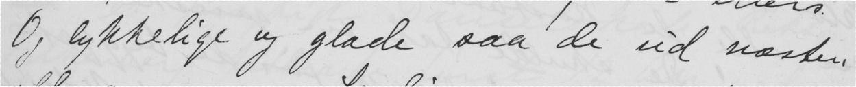Og lykkelige og glade saa de ud næsten
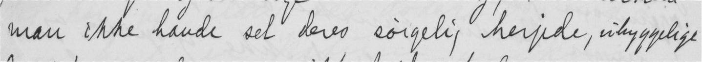man ikke havde set deres sørgelig herjede, uhyggelige
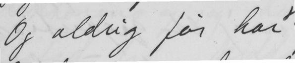Og aldrig før har
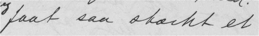faat saa stærkt et
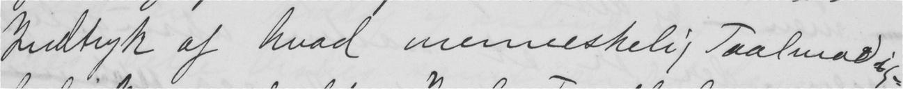Indtryk af hvad menneskelig Taalmodig-
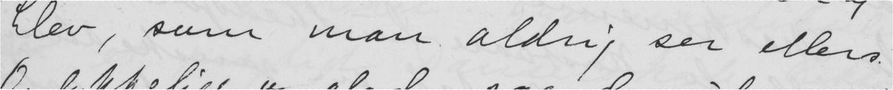Elev, som man aldrig ser ellers.
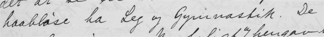haabløse ha Leg og Gymnastik. De
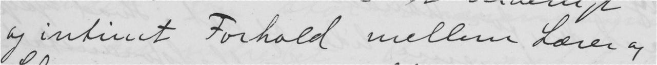og intimt Forhold mellem Lærer og
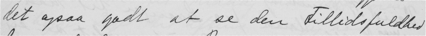det ogsaa godt at se den Tillidsfuldhed
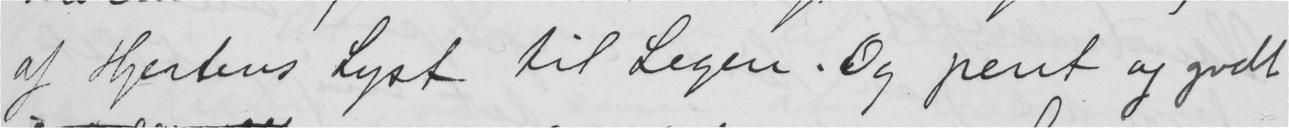af Hjertens Lyst til Legen. Og pent og godt
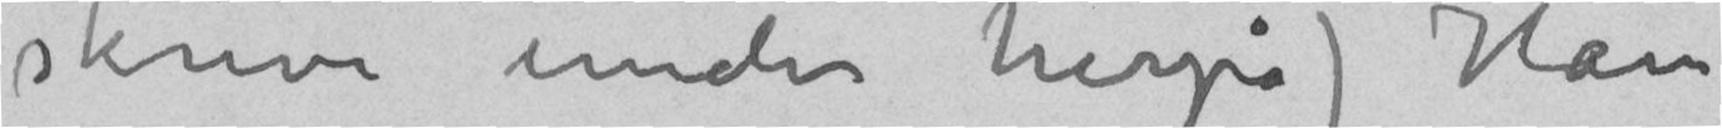skrive under herpå) Han
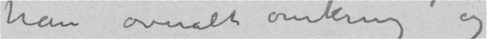han overalt omkring og
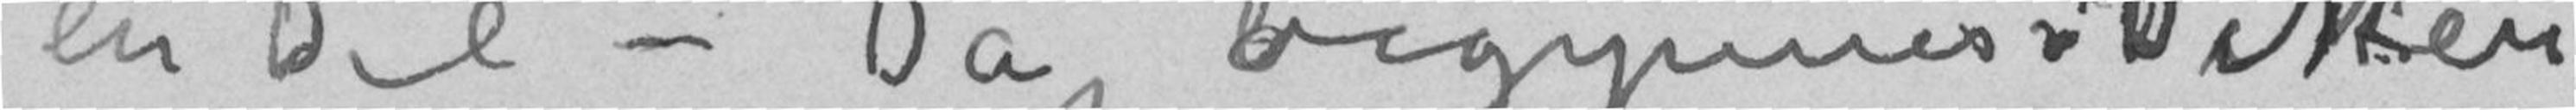en Del – Da begynner: Ditten
